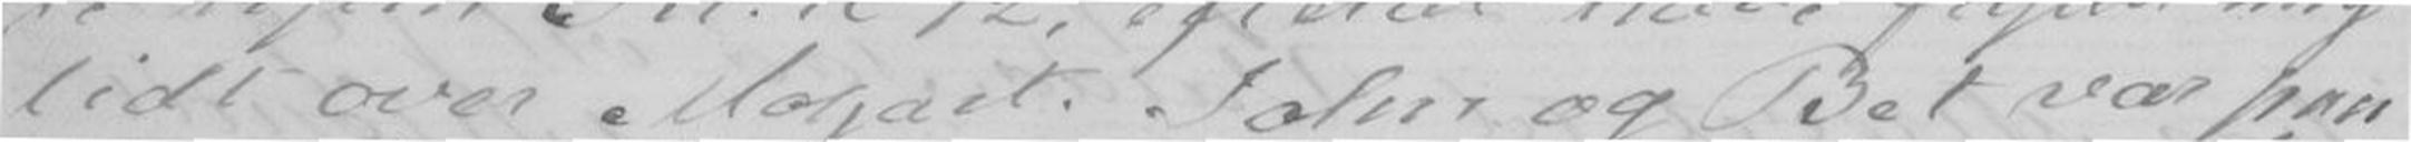lidt over Mozart. John og Bet var paa
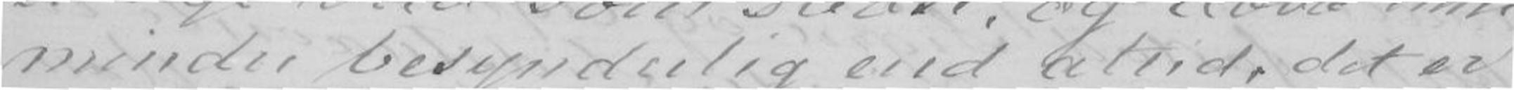mindre besynderlig end altid, det er
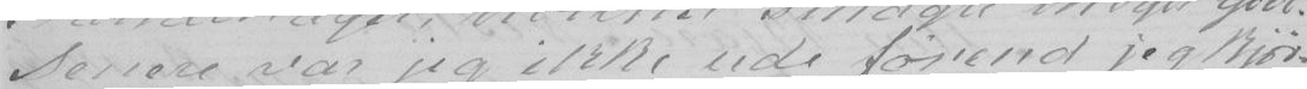Senere var jeg ikke ude førend jeg kjør-
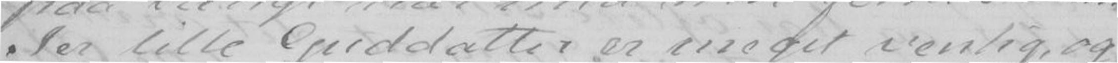Jer lille Guddatter er meget venlig, og
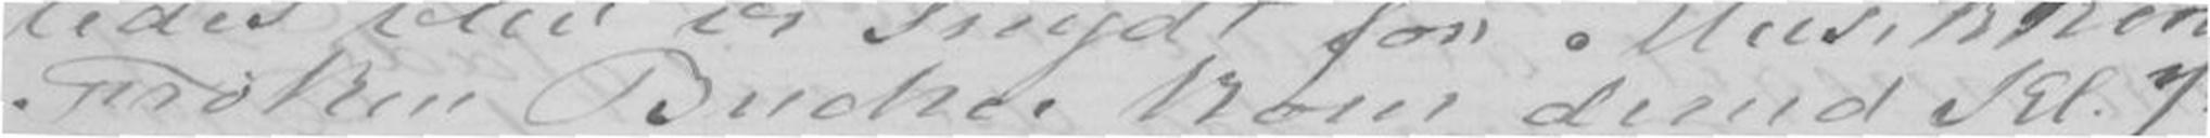Frøken Bucher kom derud Kl.7.
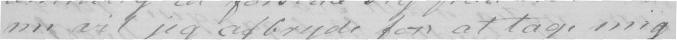nu vil jeg afbryte for at tage mig
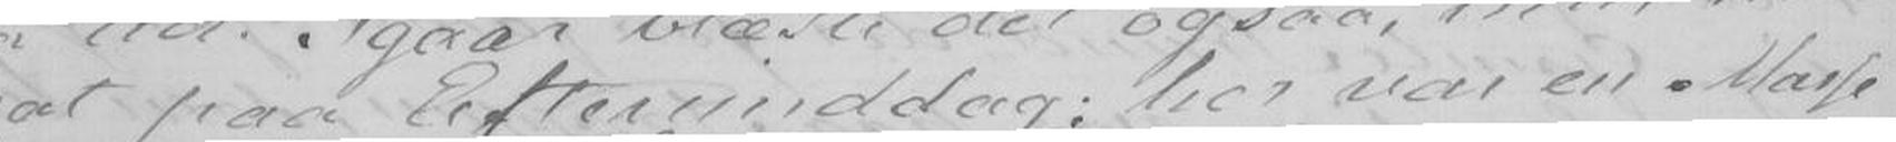at paa Eftermiddag; her var en Masse
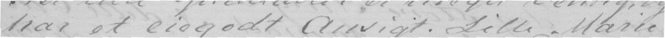har et eiegodt Ansigt. Lille Marie
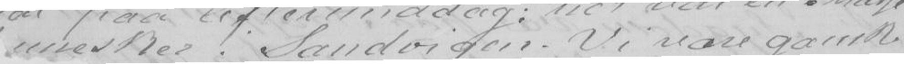nnesker i Sandvigen. Vi vare ganske
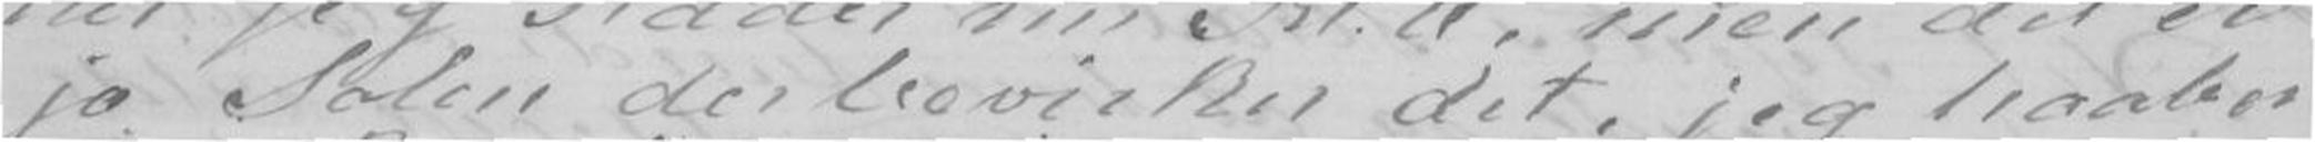jo John der bevirker det, jeg haaber
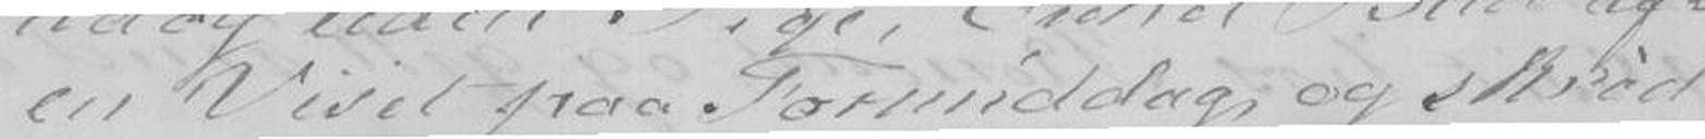en Visit paa Formiddag, og skrød
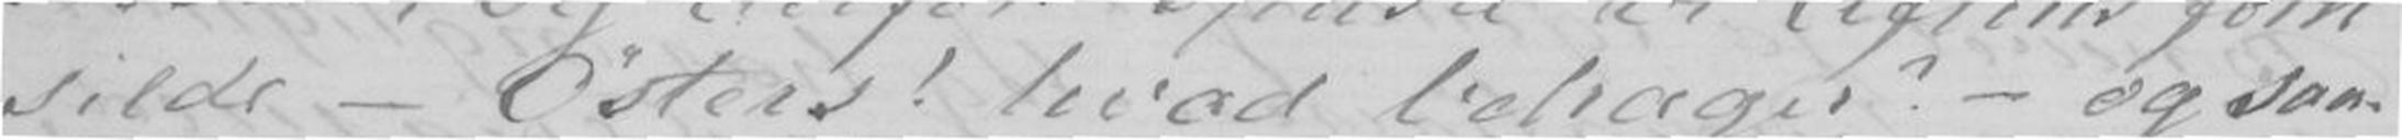silde - Østers! hvad behager? - og saa-
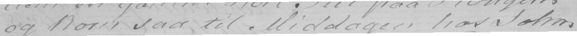og kom saa til Middagen hos John
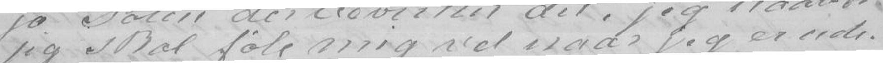jeg skal føle mig vel naar jeg er ude.
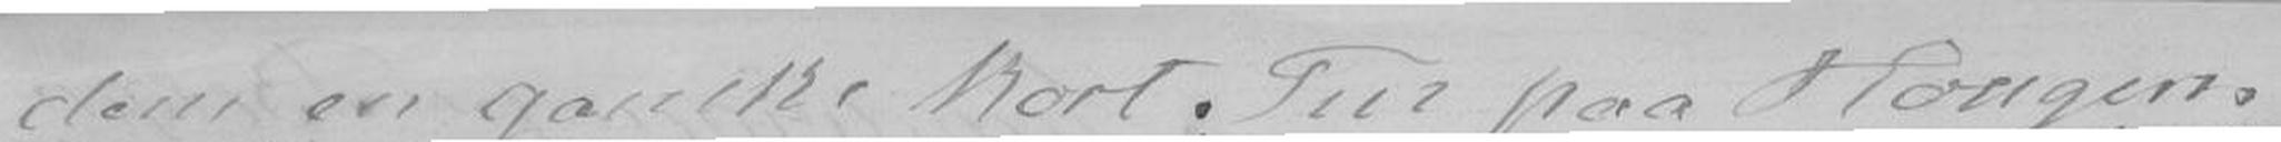dem en ganske Kort Tur paa Hougen,
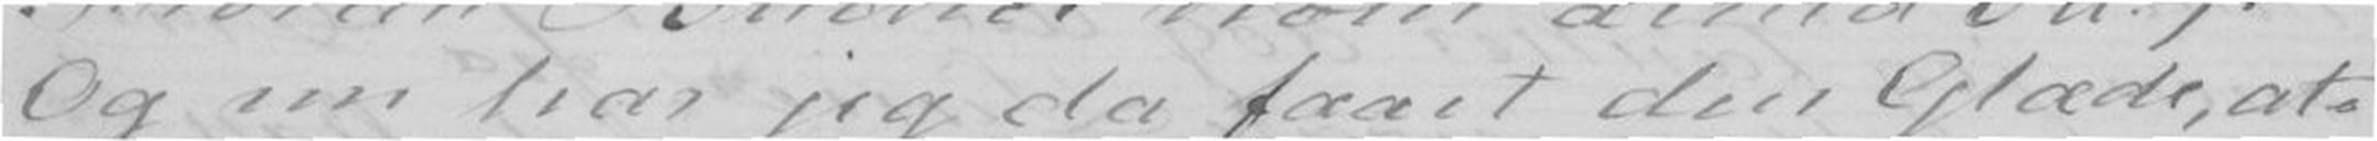Og nu har jeg da faaet den Glæde, at-
